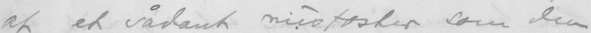af et sådant misfoster som den
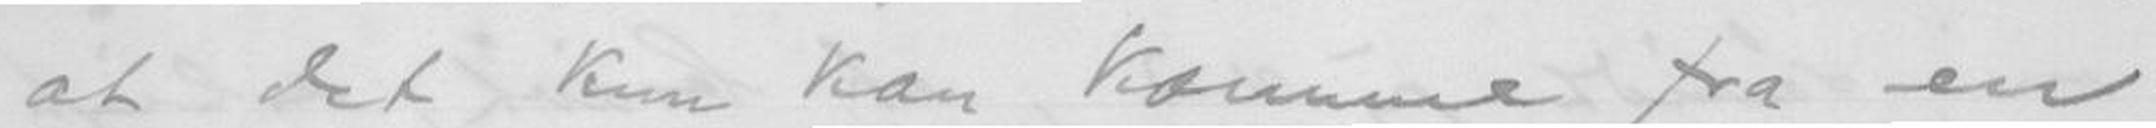at det kun kan komme fra en
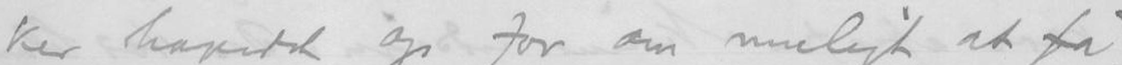ker hovedet op for om muligt at få
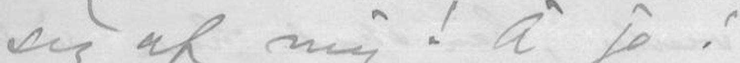sig af mig! Å jo!
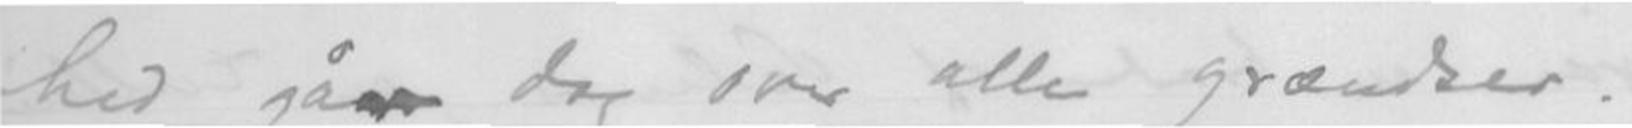hed går dog over alle grændser.
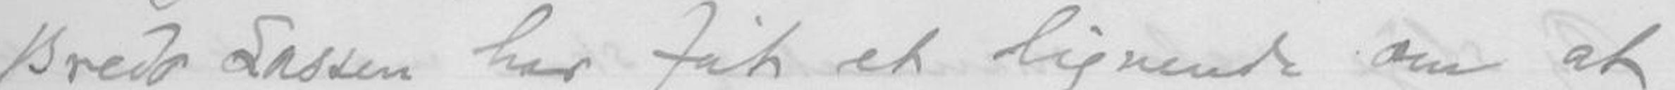Brede Lassen har fåt at lignende om at
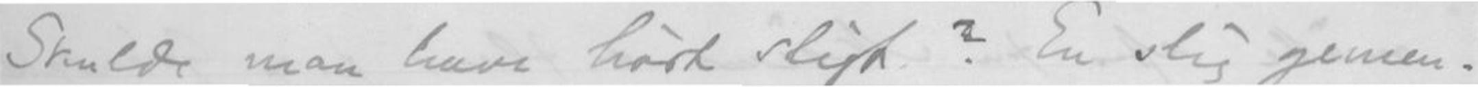Skulde man have hørt sligt? En slig gemen-
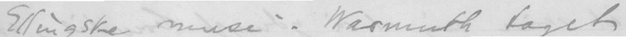Ellingske muse". Warmuth taget
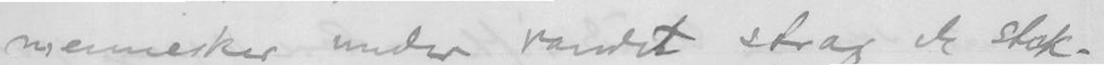mennesker under vandet strax de stik-
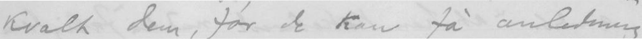kvalt dem, før de kan få anledning
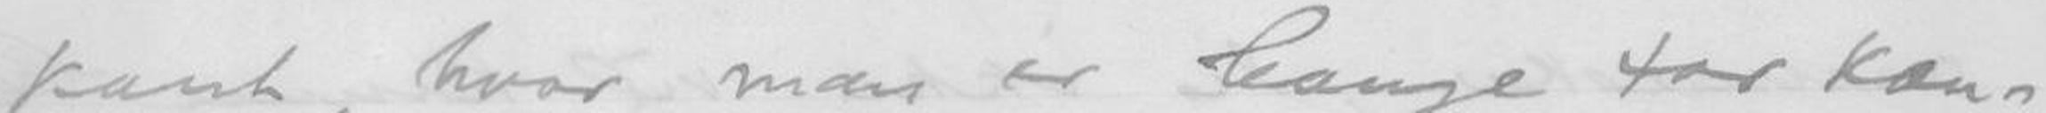kant, hvor man er bange for kon-
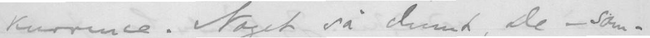kurrance. Noget så dumt, De - som
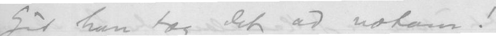Gid han tog det ad notam!
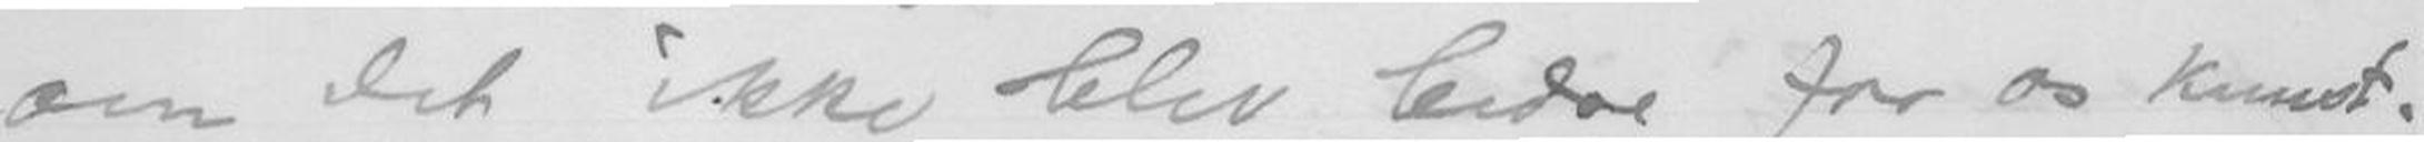om det ikke blev bedre for os kunst-
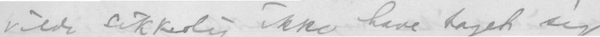vilde sikkerlig ikke have taget sig
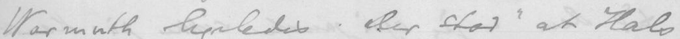Warmuth ligeledes der stod "at Hals
